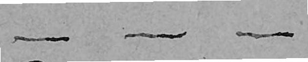- - -
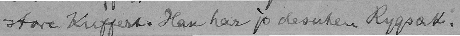store Kuffert. Han har jo desuten Rygsæk.
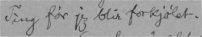Ting før jeg blir forkjølet.
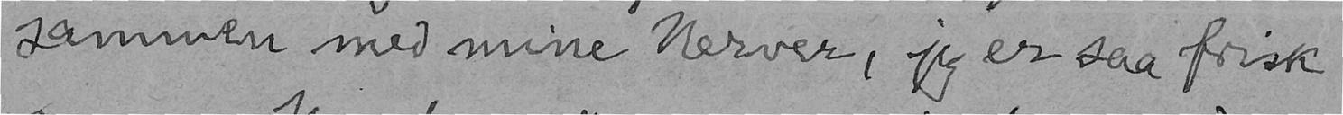sammen med mine Nerver, jeg er saa frisk
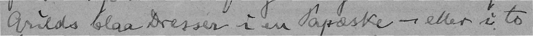Arilds blaa Dresser i en Papæske - eller i to
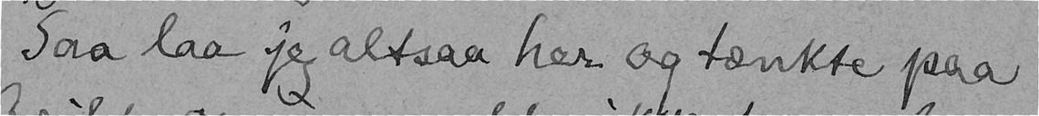Saa laa jeg altsaa her og tænkte paa
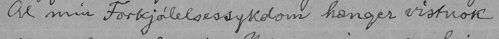Al min Forkjølelsessykdom hænger vistnok
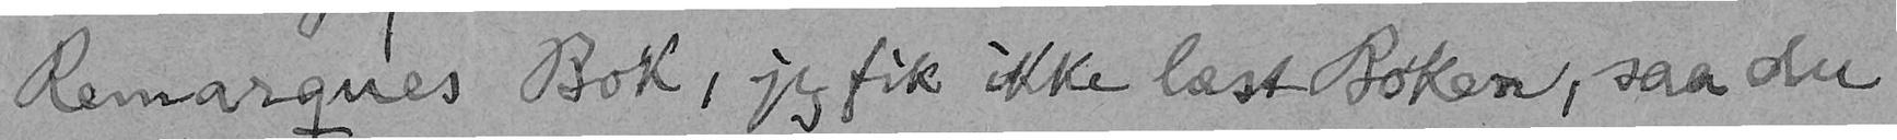Remarques Bok, jeg fik ikke læst Boken, saa du
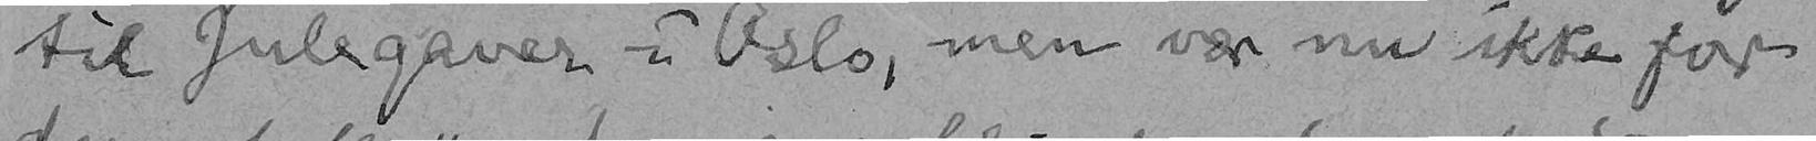til Julegaver i Oslo, men vær nu ikke for
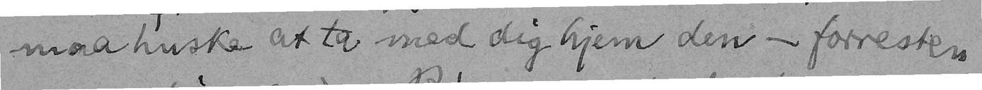maa huske at ta med dig hjem den - forresten
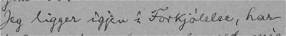Jeg ligger igjen i Forkjølelse, har
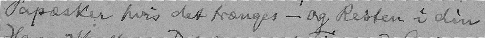Papæsker hvis det trænges - og Resten i din
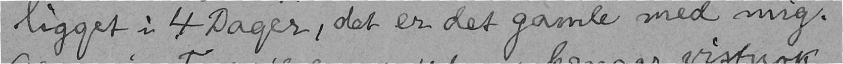ligget i 4 Dager, det er det gamle med mig.
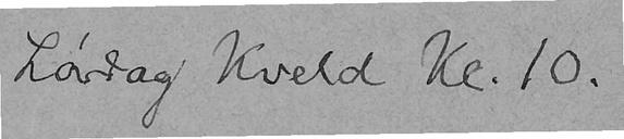Lørdag Kveld Kl. 10.
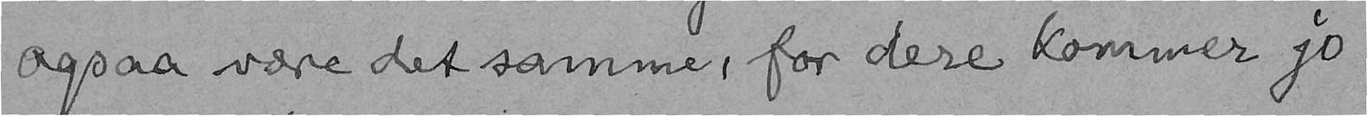ogsaa være det samme, for dere kommer jo
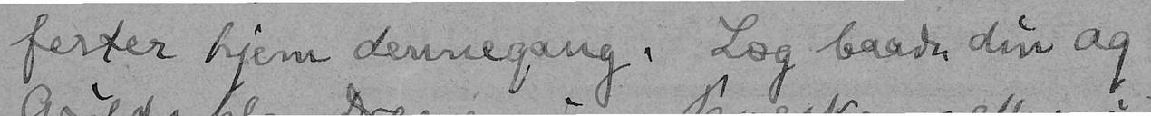ferter hjem dennegang. Læg baade din og
